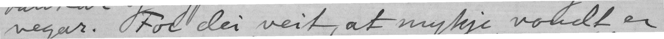vegar. For dei veit, at mykje vondt er
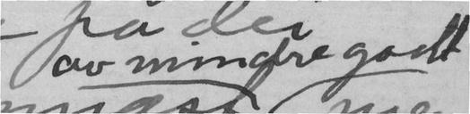av mindre godt
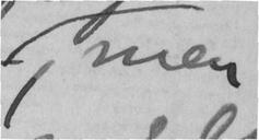men
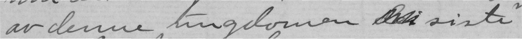av denne ungdomen dei siste
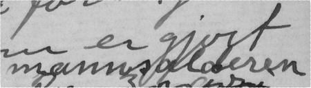mannsalderen
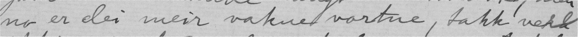no er dei meir vakne vortne, takk verd
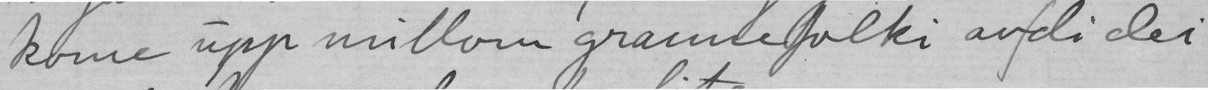kome upp millom grannefolki av di dei
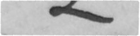2
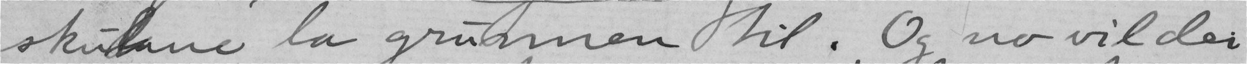skulane la grunnen til. Og no vil dei
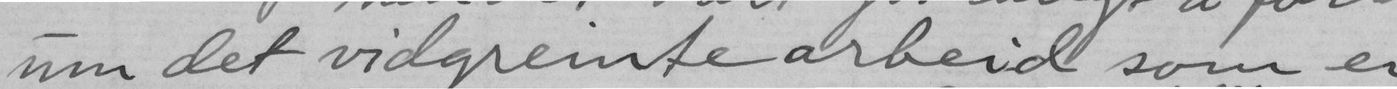um det vidgreinte arbeid som er gjort
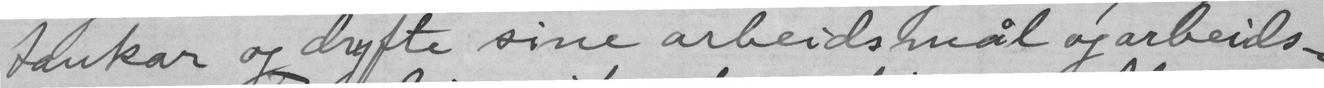tankar og dryfte sine arbeidsmål og arbeids-
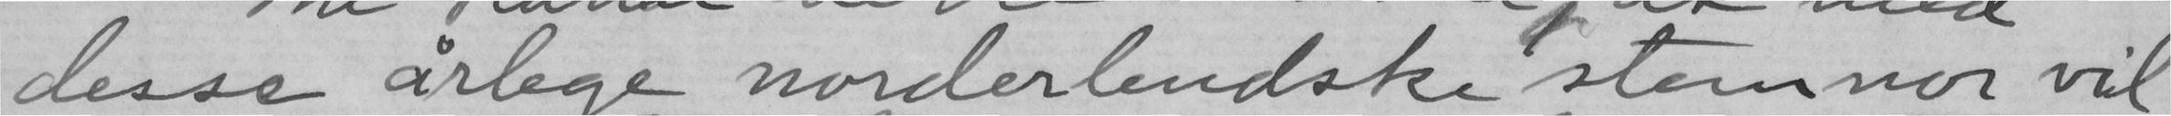desse årlege norderlendske stemner vil
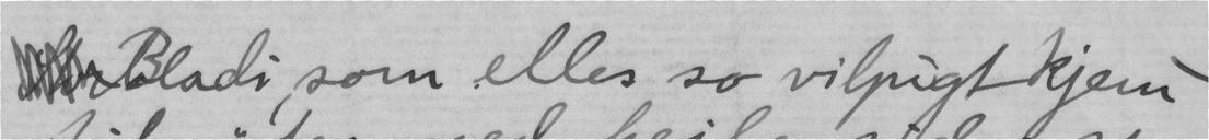Bladi, som eller so vilpigt kjem
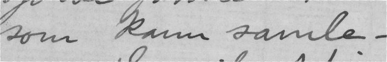som kann samle -
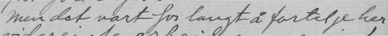Men det vart for langt å fortelje her
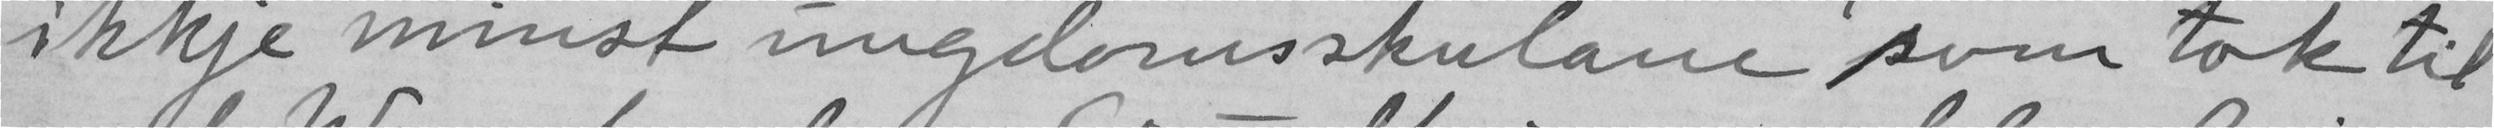ikkje minst ungdomsskulane som tok til
